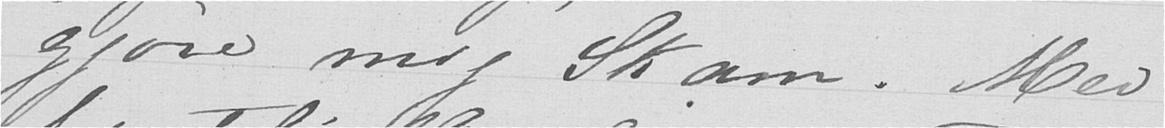gjøre mig Skam. Med
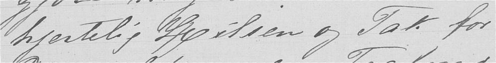hjertelig Hilsen og Tak for
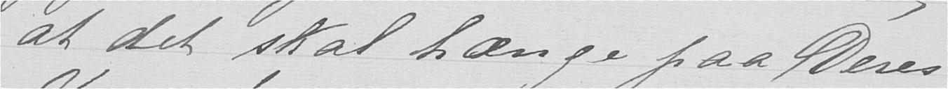at det skal hænge paa Deres
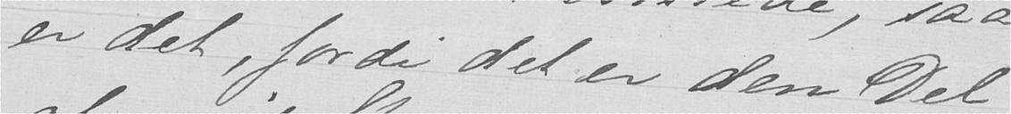er det, fordi det er den Del
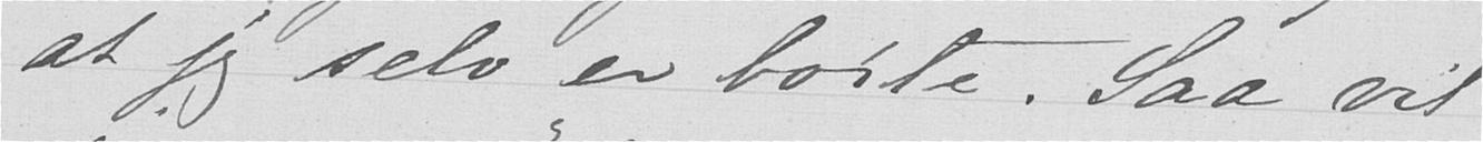at jeg selv er borte. Saa vil
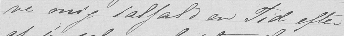ve mig ialfald en Tid efter
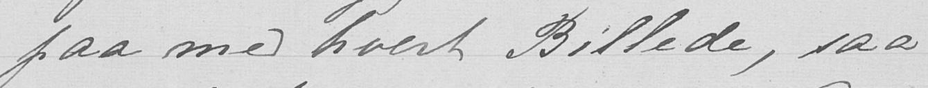paa med hvert Billede, saa
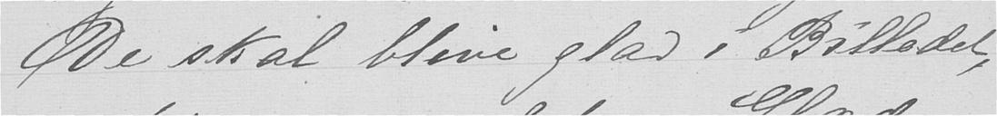De skal blive glad i Billedet,
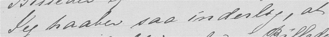Jeg haaber saa inderlig, at
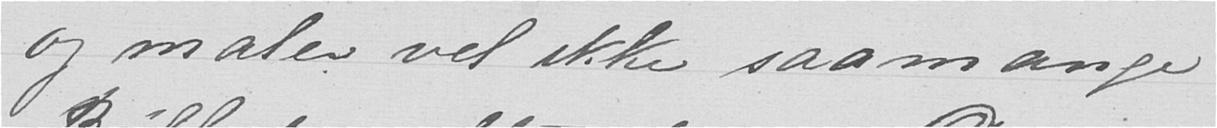og maler vel ikke saamange
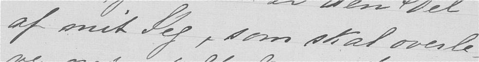af mit Jeg, som skal overle-
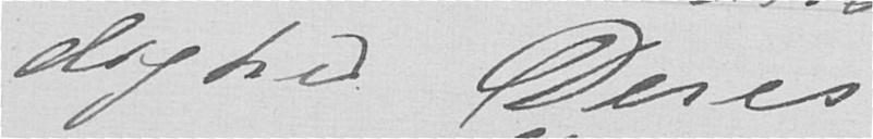dighed. Deres
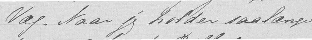Væg. Naar jeg holder saa længe
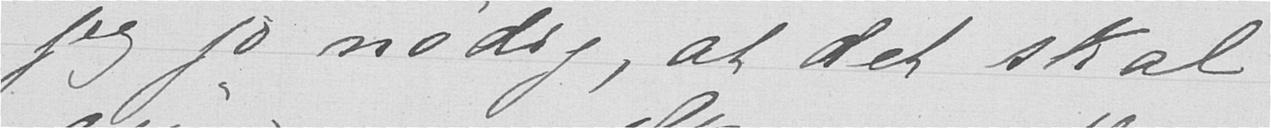jeg jo nødig, at det skal
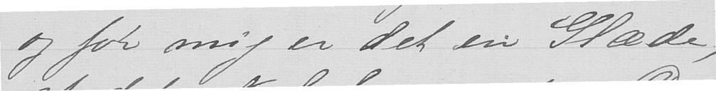og for mig er det en Glæde,
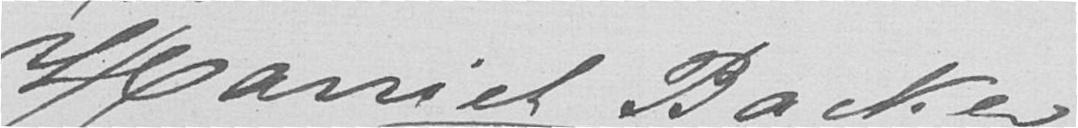Harriet Backer
